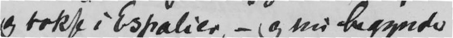og vokse i Espalier, - og nu begynde
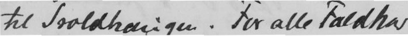til Troldhaugen. For alle Fald har
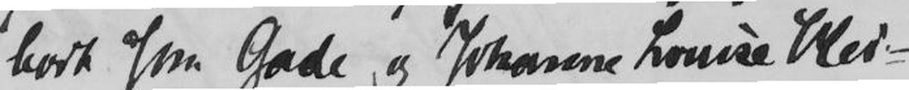bort som Gade og Johanne Louise Wei-
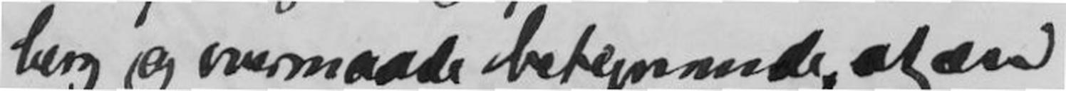berg og overmaade betejnende at en
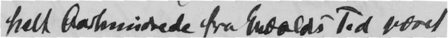helt Aarhundrede fra Ewalds Tid været
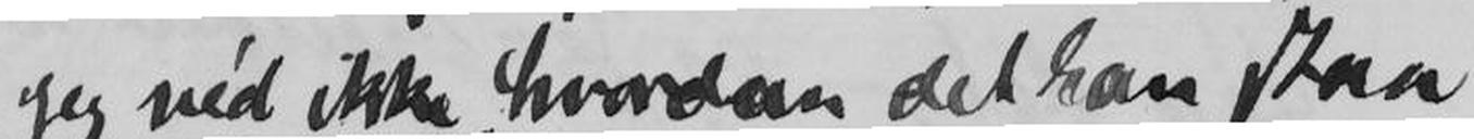jeg ved ikke hvordan det kan staa
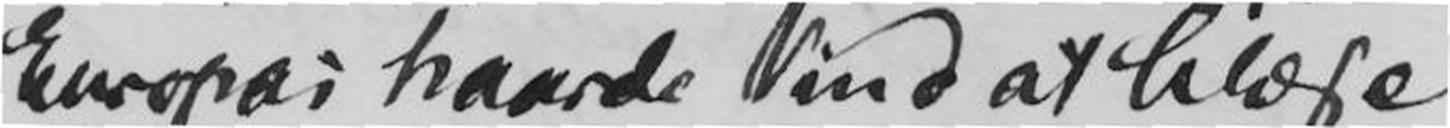Europa i haarde Vind at blæse
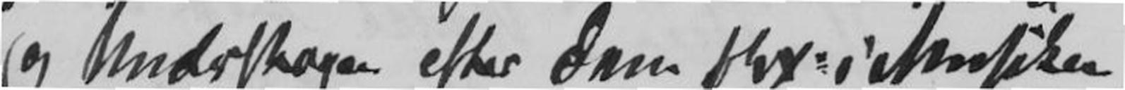og Underskogen efter dem fex: i Musiken,
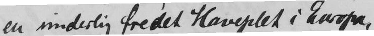en inderlig fredet Haveplet i Europa,
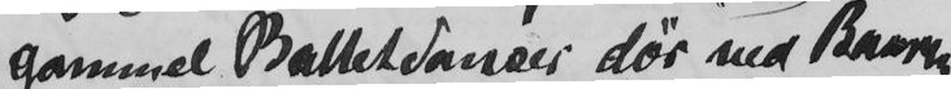gammel Balletdanser dør ved Baaren.
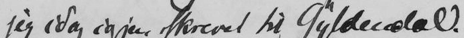jeg idag igjen skrevet til Gyldendal.
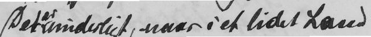Det er underligt, naar i et lidet Land
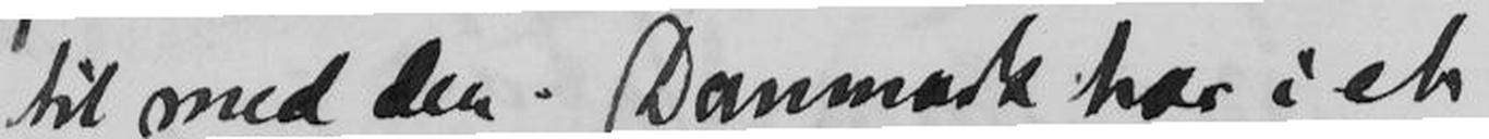til med den. Danmark har i et
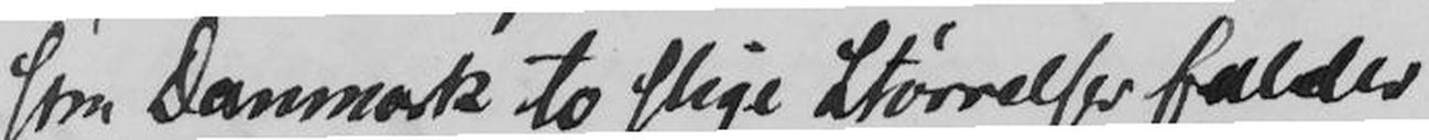som Danmark to slige Størrelser falder
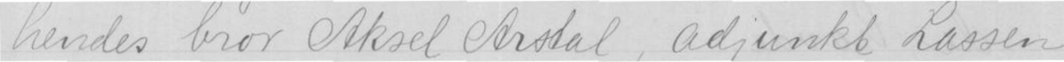hendes bror Aksel Arstal, adjunkt Lassen
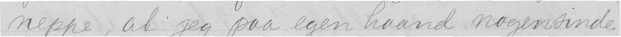neppe, at jeg paa egen haand nogensinde
